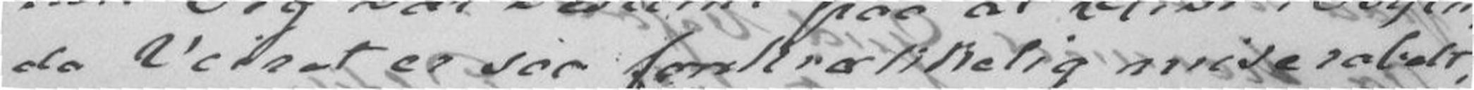da Veiret er saa forskrækkelig miserabelt,
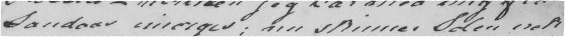Landaas imorgen; nu skinner Solen nok
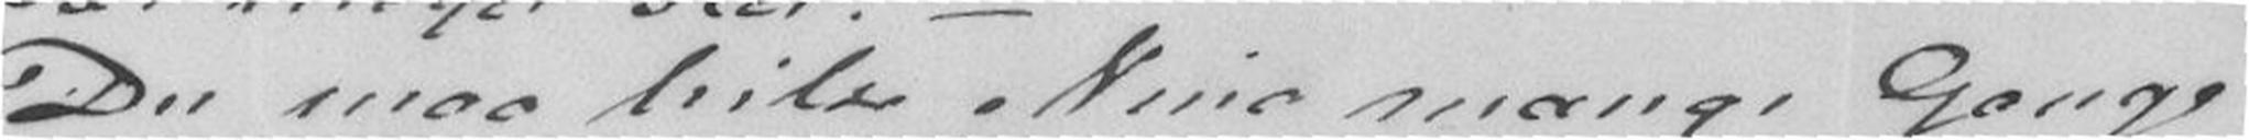Du maa hilse Nina mangen Gange
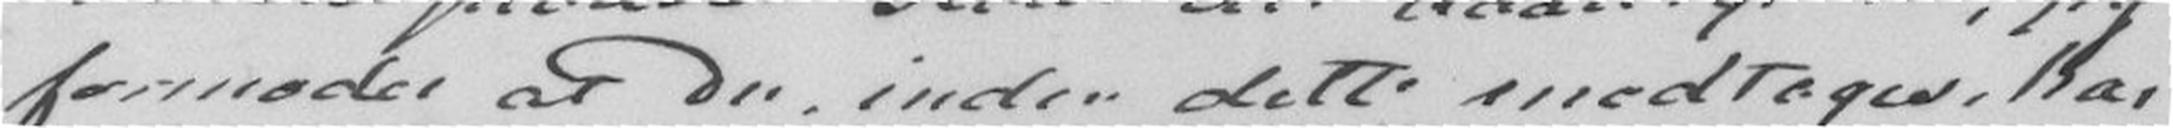formoder at Du inden dette modtages, har
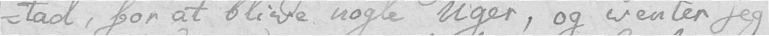-stad, for at blive nogle Uger, og venter jeg
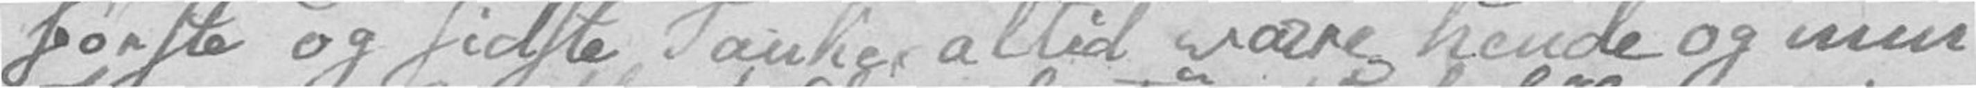første og sidste Tanke, altid være hende og min
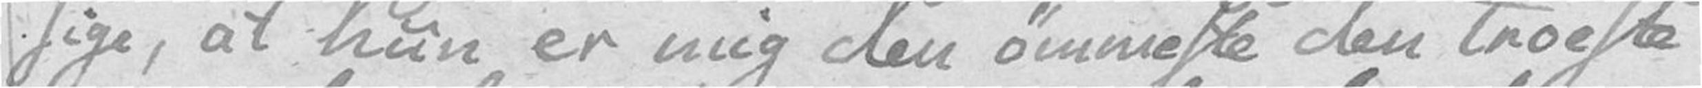sige, at hun er mig den ømmeste den troeste
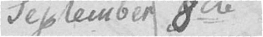September 8de
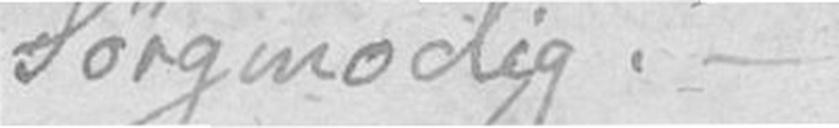Sørgmodig. –
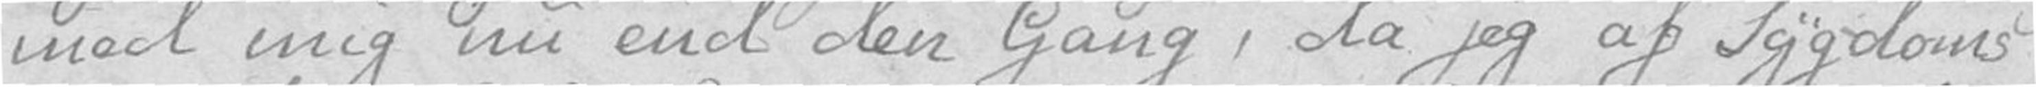med mig nu end den Gang, da jeg af Sygdoms
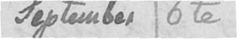September 6te
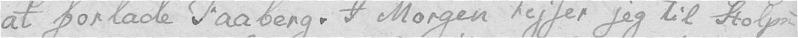at forlade Faaberg. I Morgen rejser jeg til Stolpe
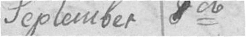September 7de
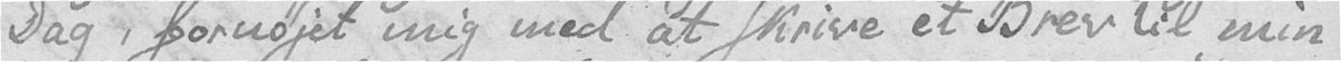Dag, fornøjet mig med at skrive et Brev til min
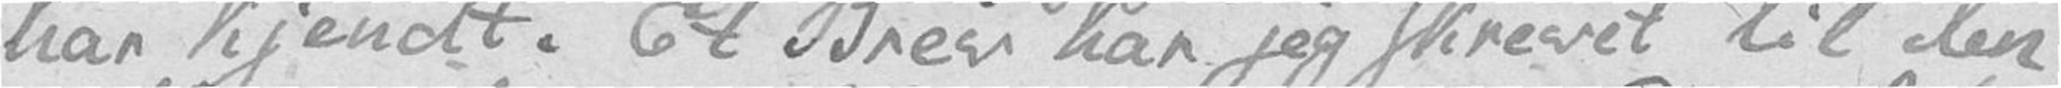har kjendt. Et Brev har jeg skrevet til den
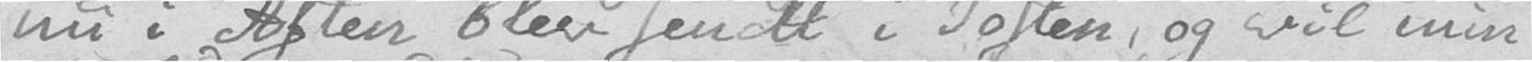nu i Aften blev sendt i Posten, og vil min
